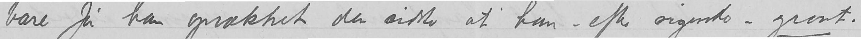bare før han opvækket den sidste at han – efter sigende – graat.
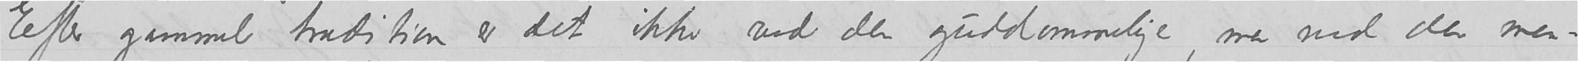Efter gammel tradition er det ikke ved den guddommelige, men ved den men-
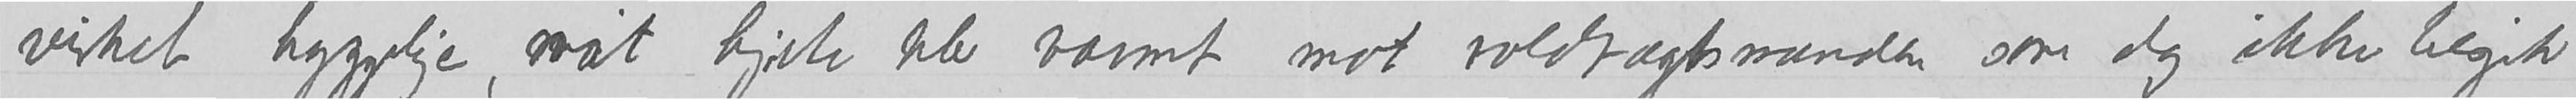virket hyggelige, vårt hjerte ble varmt mot voldtægtsmanden som dog ikke begik
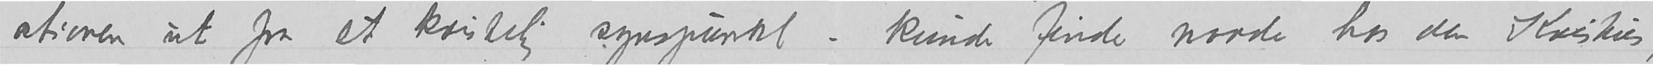ationen ut fra et kristelig synspunkt – kunde finde naade hos den Kristus, som ganske vist
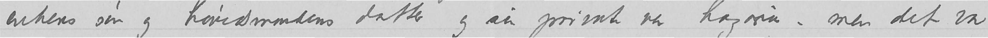enkens søn og høvedmandens datter og sin private ven Lazaru, men det var
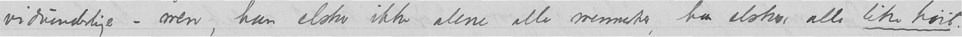vidunderlige – men, han elsker ikke alene alle mennesker, han elsker alle like høit.
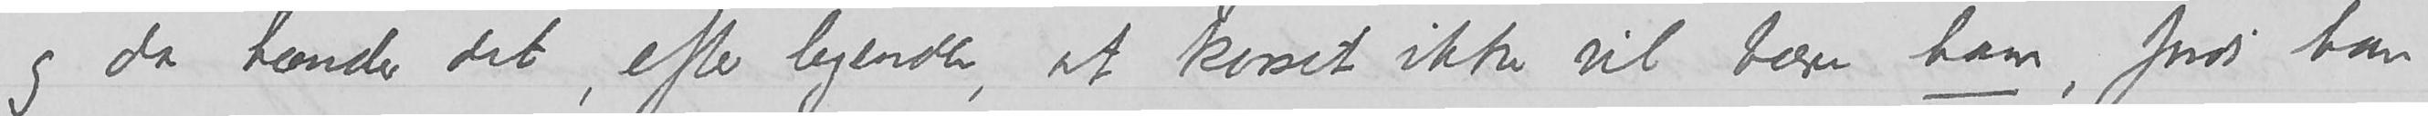– og da hænder det, efter legender, at korset ikke vil bære ham, fordi han
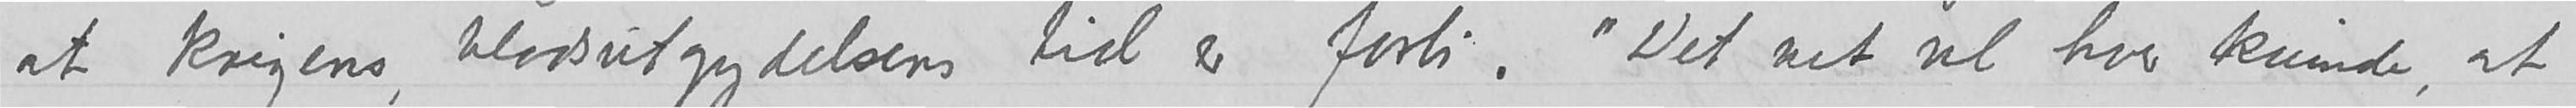at krigens, blodsutgydelsens tid er forbi.  «Det vet vel hver kvinde, at
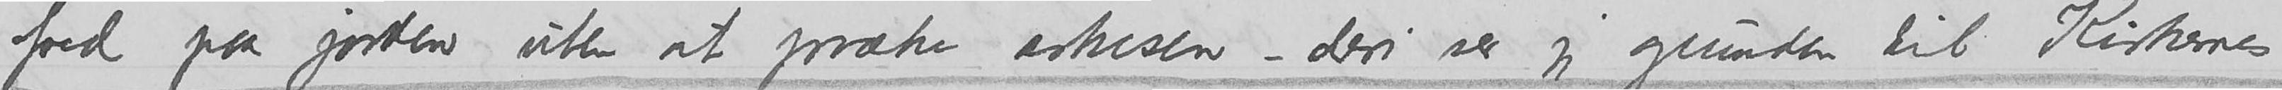fred på jorden uten at præke askesen – deri ser jeg grunden til Kirkernes
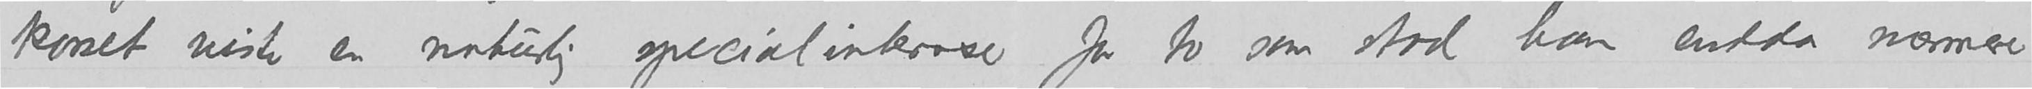korset viste en naturlig specialinteresse for to som stod ham endda nærmere
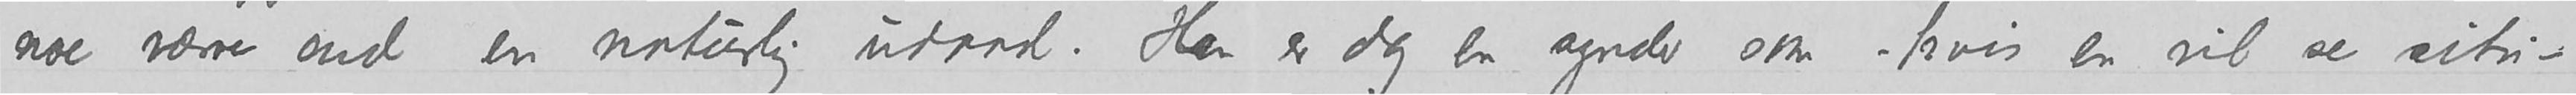noe værre end en naturlig udaad.  Han er dog en synder som – hvis en vil se situ-
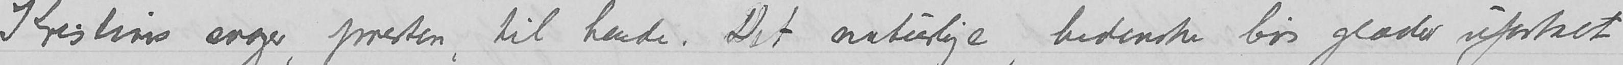Kristins svoger, presten, til hende. Det naturlige, hedenske livs glæder ufortært/talt
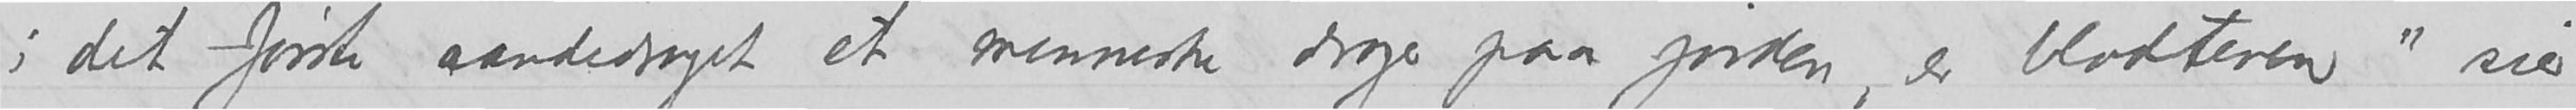i det første aandedraget et menneske drager paa jorden, er blodteven sier
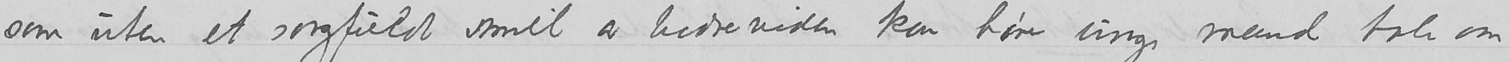som uten et sorgfuldt smil av bedreviden kan høre unge mænd tale om
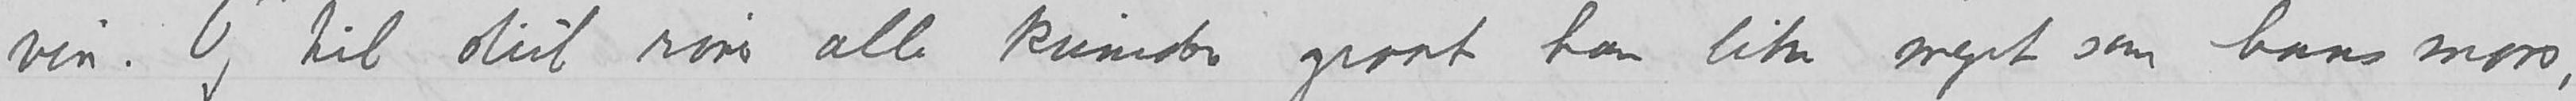Og til slut rører alle kvinders graat ham like meget som hans mors,
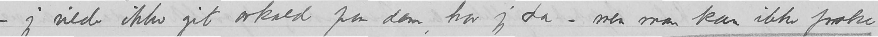–jeg vilde ikke git avkald paa dem, tror jeg da – men man kan ikke fordre
